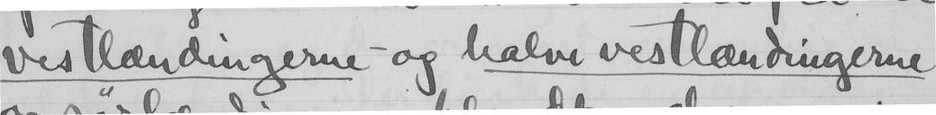vestlændingerne - og halve vestlændingerne
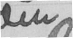den
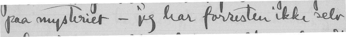paa mysteriet - jeg har forresten ikke selv
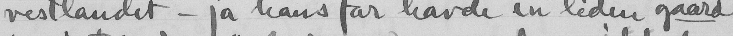vestlandet - ja hans far havde en liden gaard
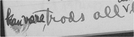kan være, trods all
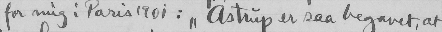for mig i Paris 1901 : "Astrup er saa begavet, at
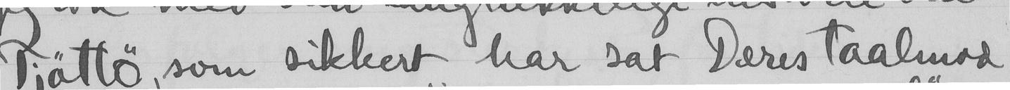Tjøttø, som sikkert har sat Deres taalmod
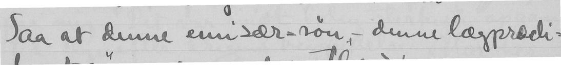Saa at denne ennsær-søn - denne lægprædi-
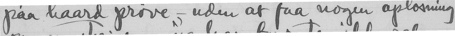paa haard prøve, - uden at faa nogen opløsning
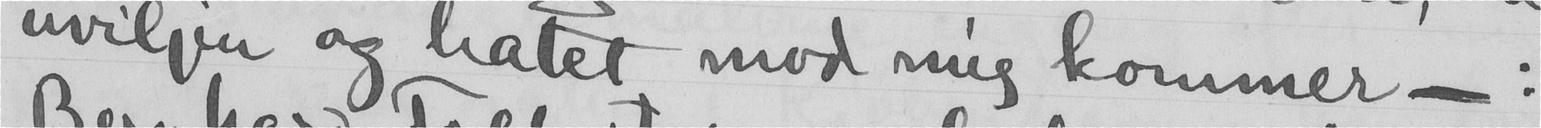uviljen og hatet mod mig kommer -:
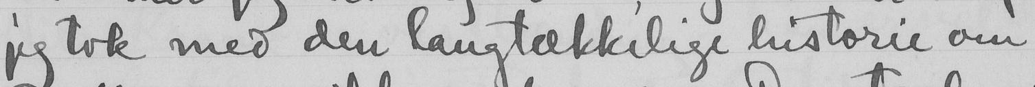jeg tok med den langtækkelige historie om
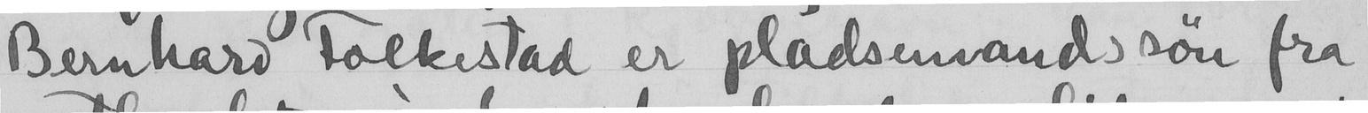Bernhard Folkestad er pladsemands søn fra
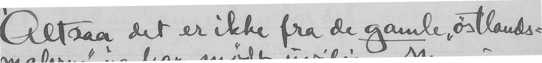Altsaa det er ikke fra de gamle "østlands-
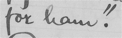for ham!!
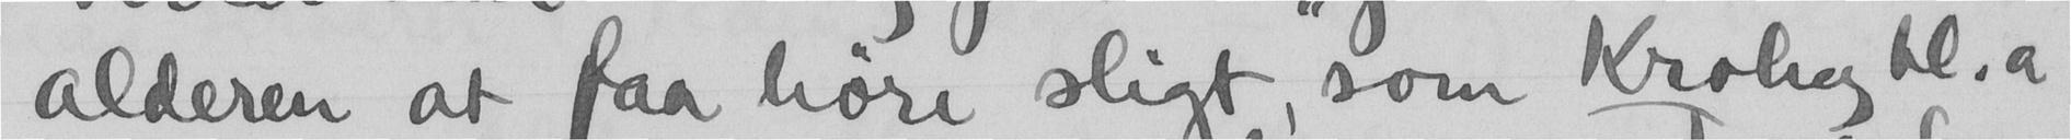alderen at faa høre sligt, som Krohg bl.a
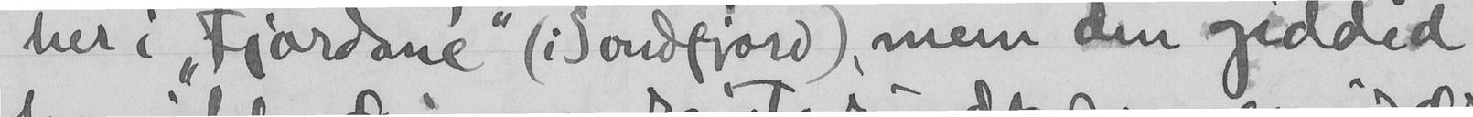her i "Fjordane" (i Sondfjord), men den gidded
